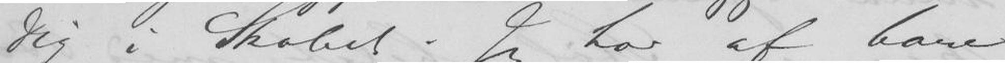Dig i Skabet. Jeg har af bare
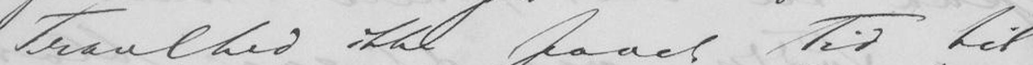Travlhed ikke faaet Tid til
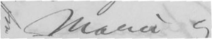Marie og
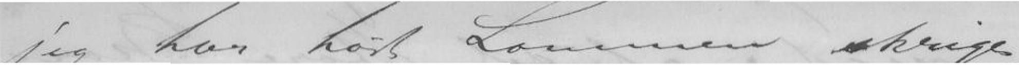jeg har hørt Lommen skrige
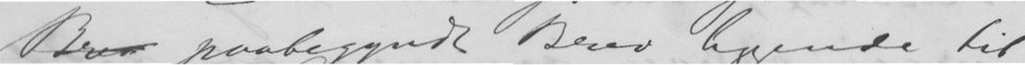Brev paabegyndt Brev liggende til
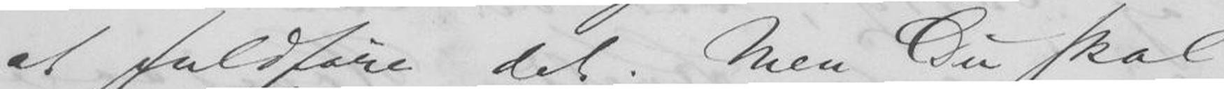at fuldføre det. Men du skal
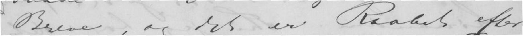Breve, og det er Raabet efter
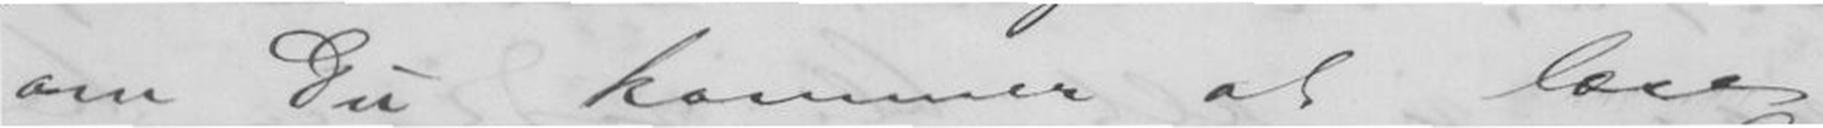om Du kommer at læse
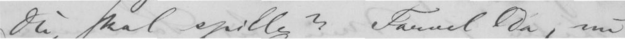du skal spille? Farvel Da, nu
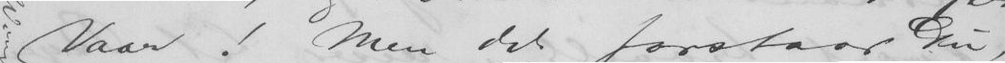Vaar! Men det forstaaar Du,
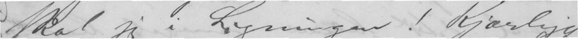skal jeg i Ligningen! Kjærlige
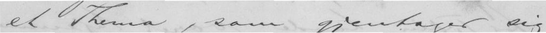et Thema, som gjentager sig
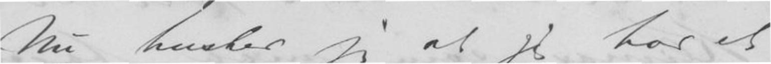- Nu husker jeg at jeg har et
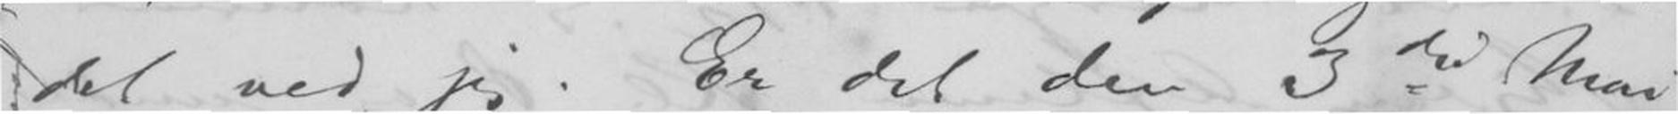det ved jeg. Er det den 3die Mai
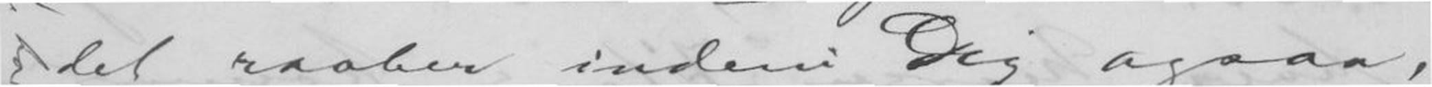det raaber indeni Dig ogsaa,
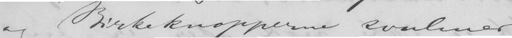og Birkeknopperne svulmer.
